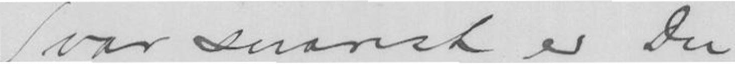Svar snarest er Du
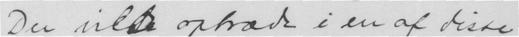Du vilde optræde i en af disse
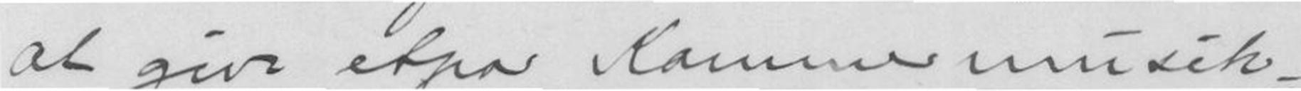at give etpar Kammermusik-
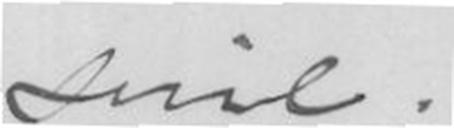snil.
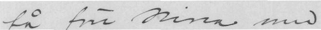få fru Nina med
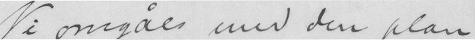Vi omgåes med den plan
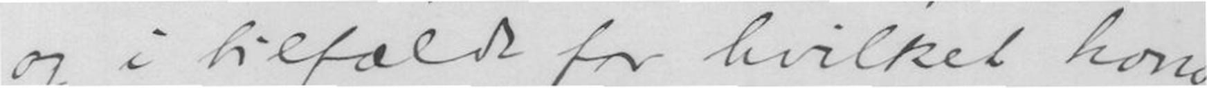og i tilfælde for hvilket hono-
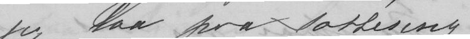jeg laa paa Sotteseng.
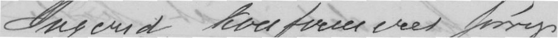Ingerid, konfirmeret forrige
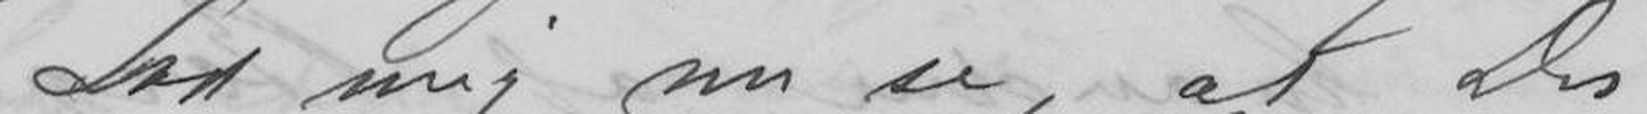Lad mig nu se, at Du
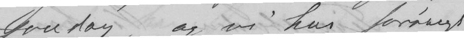Søndag, og vi har forøvrigt
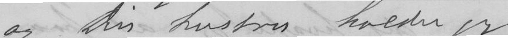og Din hustru holder jer
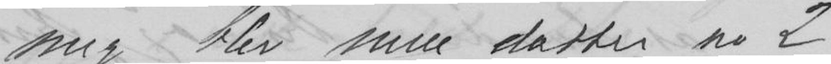mig blev min datter nr 2
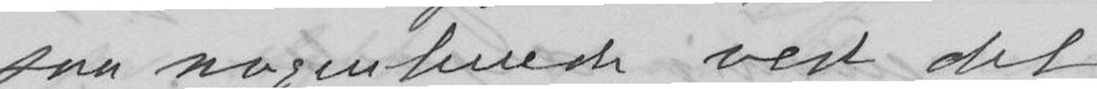saa nogenlunde ved det
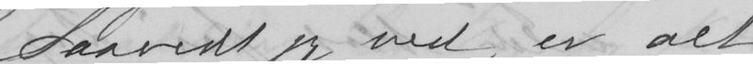Saavidt jeg ved, er alt
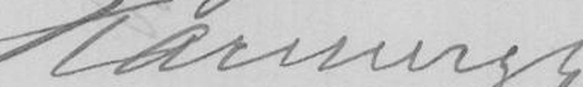Karl Bergh
